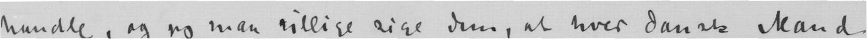handle, og jeg maa villige sige Dem, at hver dansk Mand
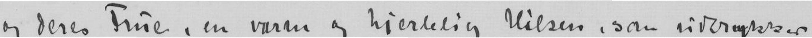og Deres Frue, en varm og hjertelig Hilsen, saa udtrykker
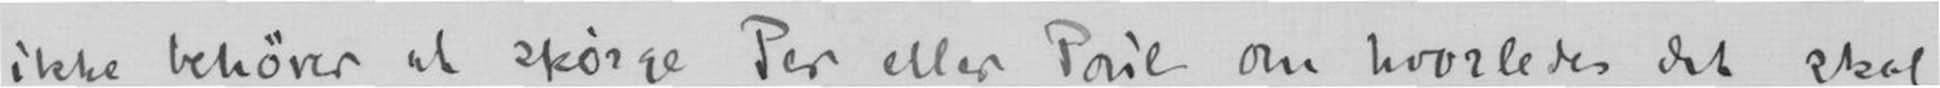ikke behøver at spørge Per eller Poul om hvorledes det skal
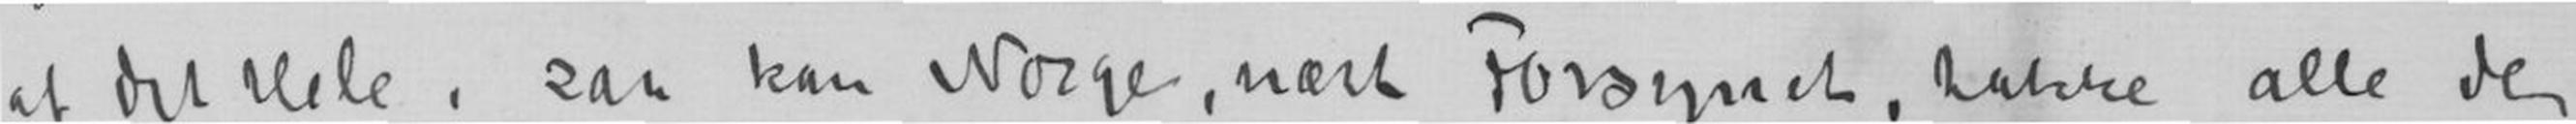af det hele, saa kan Norge, nært Forsynet, takke alle de
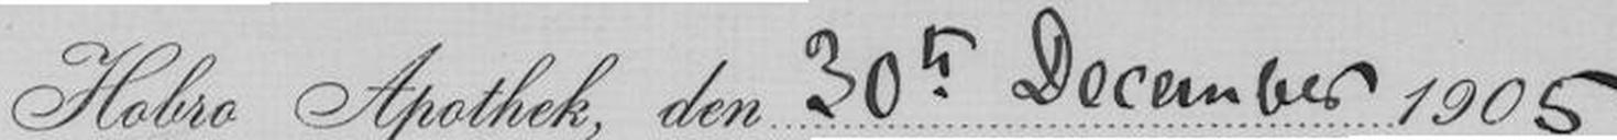Hobro Abothek, den 30te December 1905
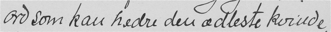Ord som kan hedre den ædleste kvinde,
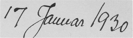17 Januar 1930
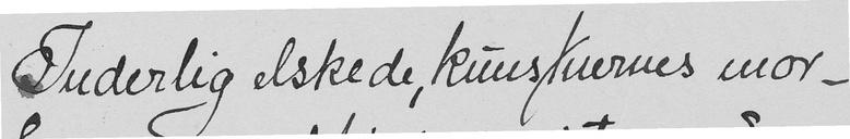Inderlig elskede, kunstnernes mor -
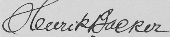Henrik Backer
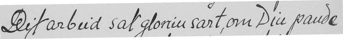Dit arbeid sat glorien sart, om Din pande
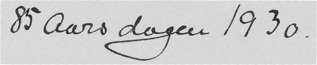85 Aars dagen 1930.
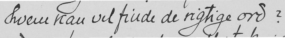hvem kan vel finde de rigtige ord?
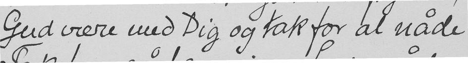Gud være med Dig og tak for al nåde
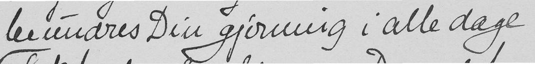beundres Din gjerning i alle dage.
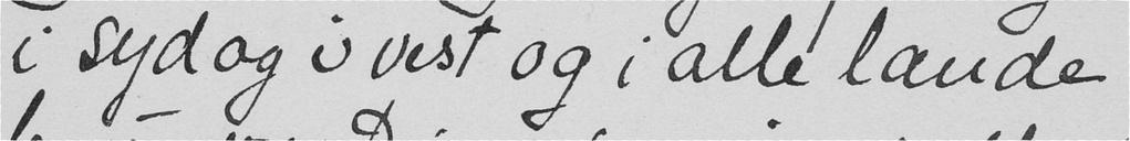i syd og i vest og i alle lande
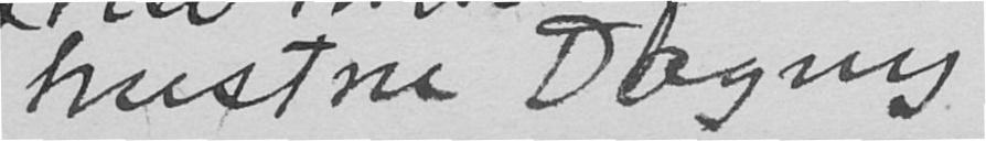hustru Dagny
